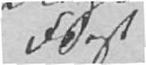Først
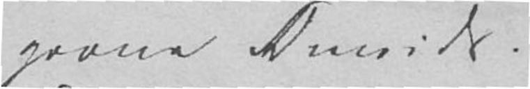grove Omrids.
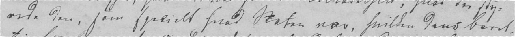rede den, som specielt hvad Staten var, hvilken dens Beret-
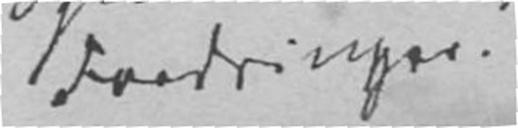Fordringer.
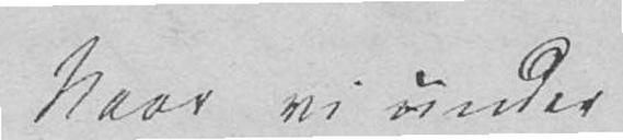Naar vi under
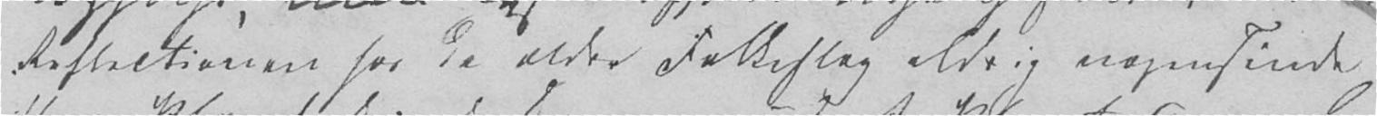Reflectionen hos de ældre Folkeslag aldrig nogensinde
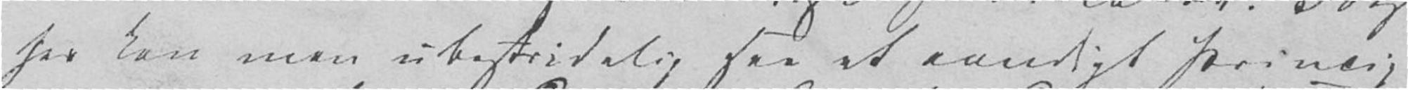her kan man ubestridelig see et aandigt Princip
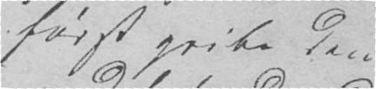først gribe den
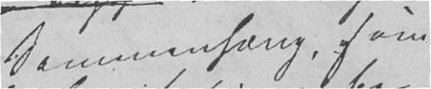Sammenhæng, som
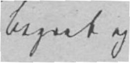Begreb og
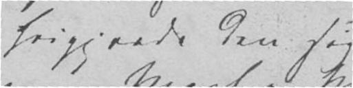frigjorde den sig
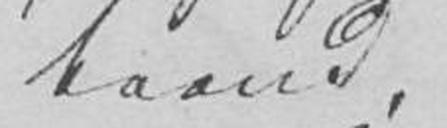baand,
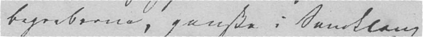begreberne, ganske i Samklang
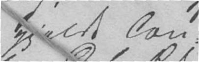gjaldt Con-
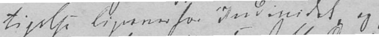tigelse ligeoverfor Individet, og
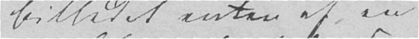Billedet enten af en
